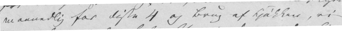maanedlig for disse 4 og Brug af Kjøkken, vi
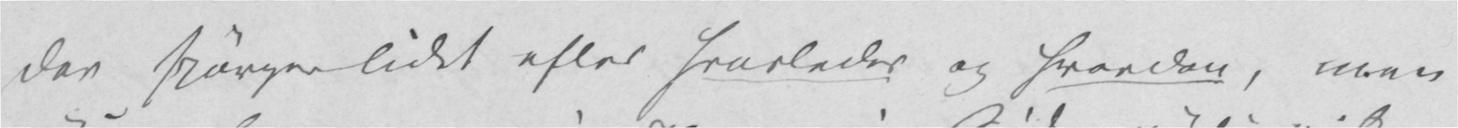der spørger lidet efter hvorledes og hvordan, men
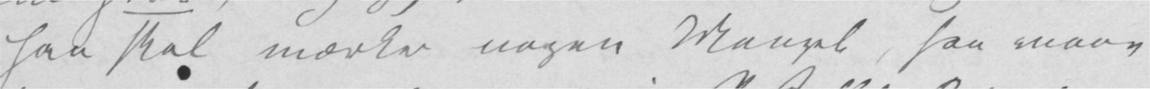han skal mærke nogen Mangel, saa maae
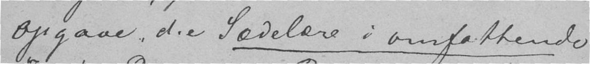opgave, d.e. Sædelære i omfattende
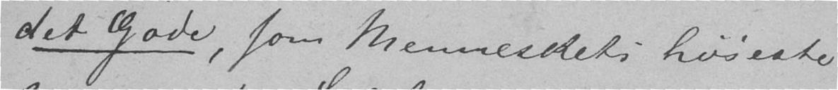det Gode, som Menneskets høieste
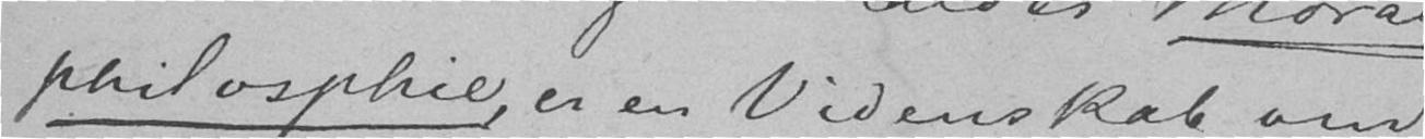philosphie, er en Videnskab om
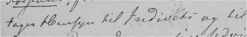tager Hensyn til Indiets og til
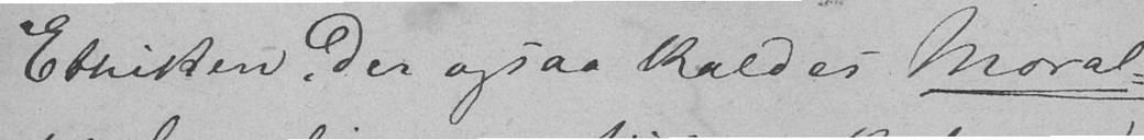Ethiken, der ogsaa kaldes Moral-
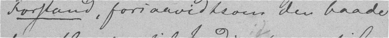Forstand, forsaavidt som den baade
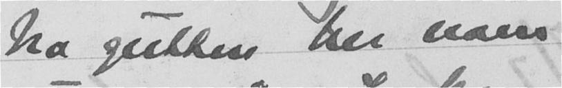ha gutten her noen
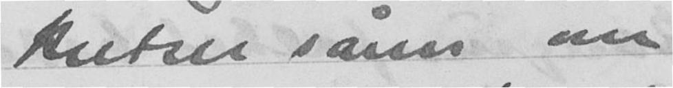kretser sånn om
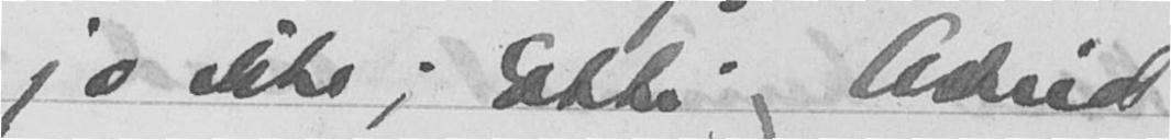jo ikke; Etti. Astrid
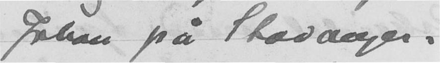Johan på Stavanger-
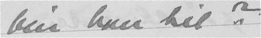blir han til?
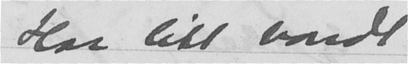Har litt vondt
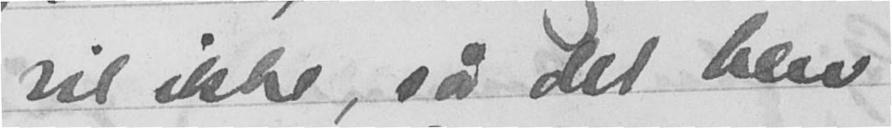vil ikke, så det blev
